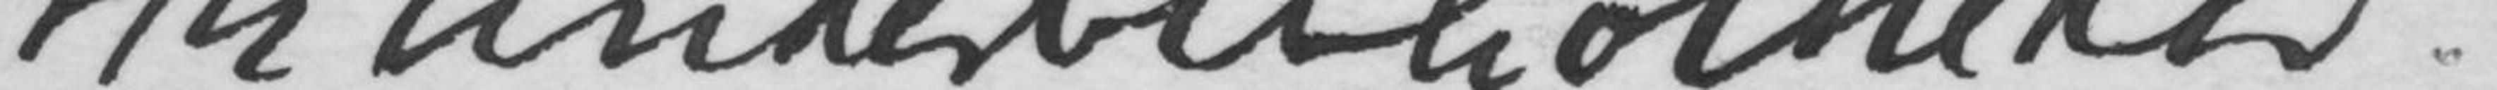Hr Underbibliothekar
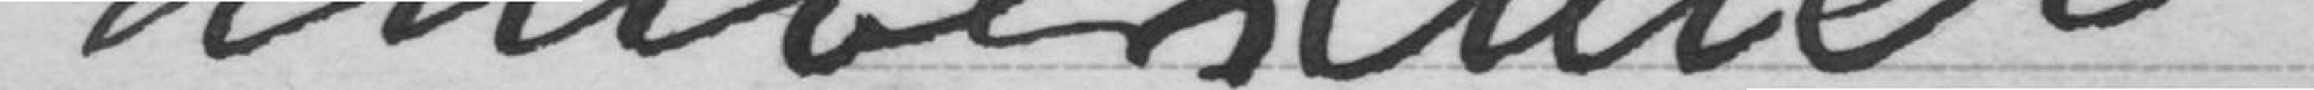Universitetet.
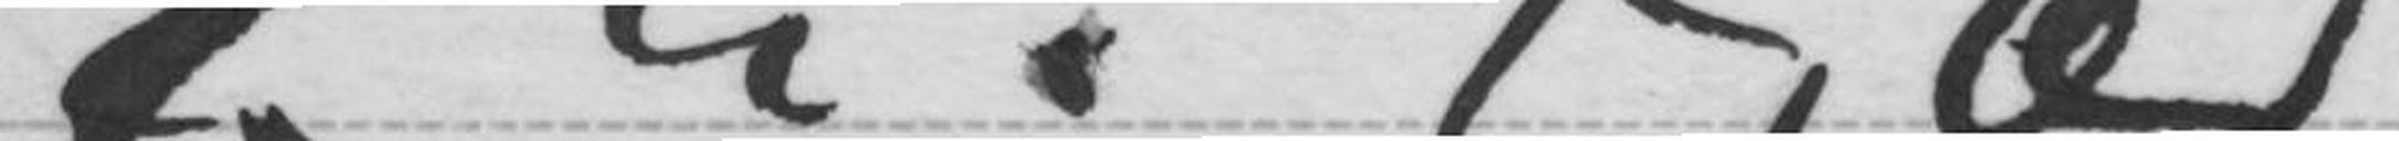A. Kjær
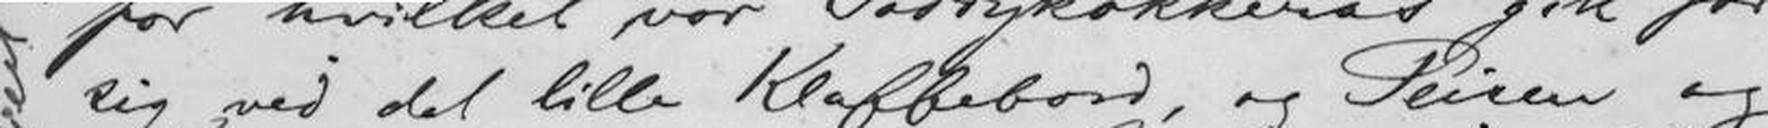sig ved det lille Klaffebord, og Peisen og
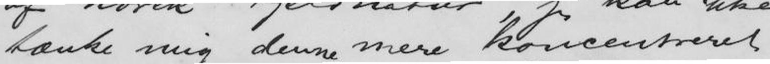tænke mig denne mere koncentreret
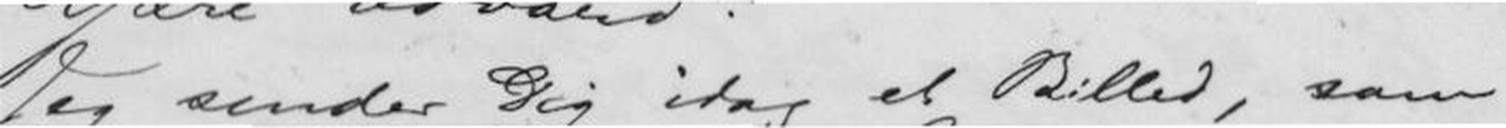Jeg sender Dig idag et Billed, som
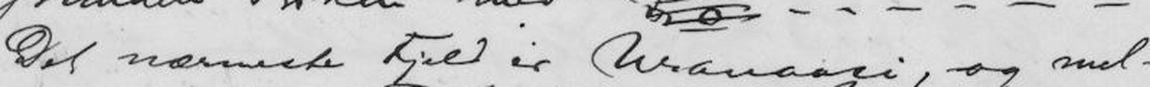Det nærmeste Fjeld er Uranaasi, og mel-
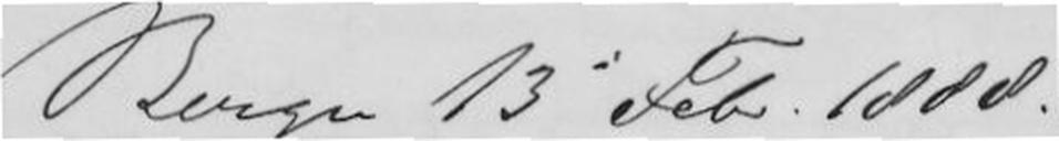Bergen 13 Feb 1888.
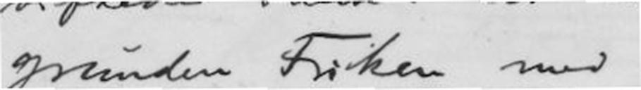grunden Friken med
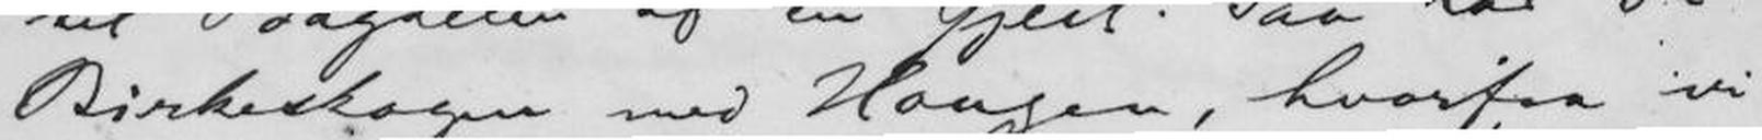Birkeskogen med Hougen, hvorfra vi
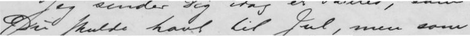Du skulde havt til Jul, men som
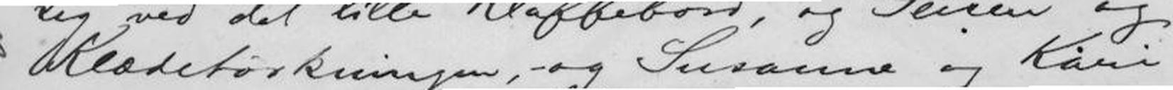Klædetørkningen, -og Susanne og Kari
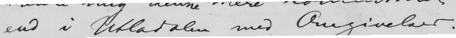end i Utladalen med Omgivelser.
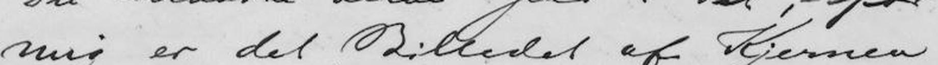mig er det Billedet af Kjernen
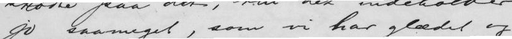jo saameget, som vi har glædet og
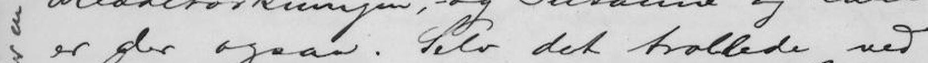er der ogsaa. Selv det trollede ved
